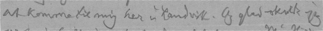at komme til mig her i Landvik. Og glad skulde jeg
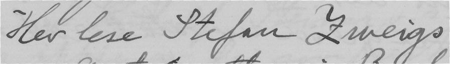Hev lese Stefan Zweigs
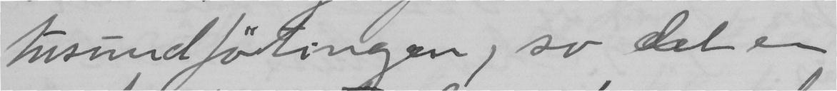tusundförtingen, so det er
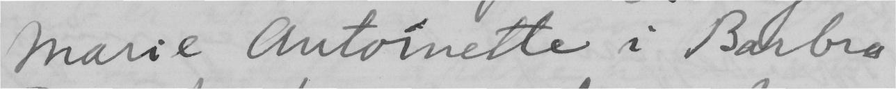Marie Antoinette i Barbra
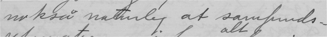nokså naturleg at samfunds-
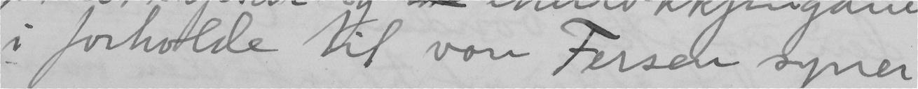i forholde til von Fersen syner
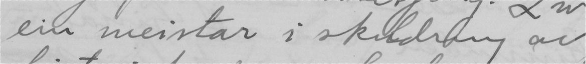ein meistar i skildring av
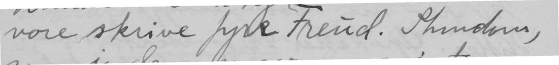vore skrive fyrr Freud. Stundom,
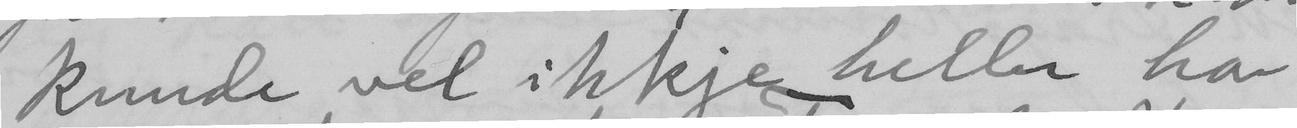kunde vel ikkje heller ha
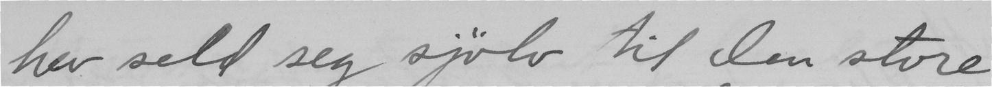hev seld seg sjölv til den store
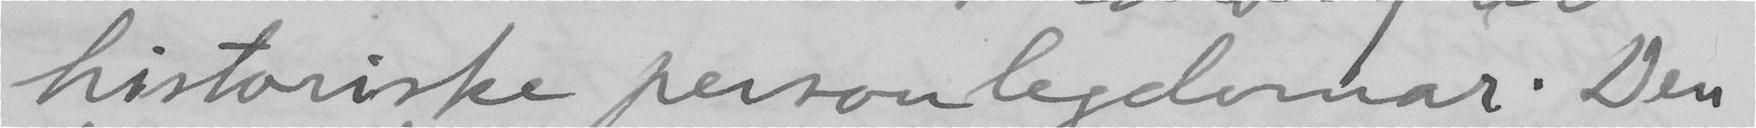historiske personlegdomar. Den
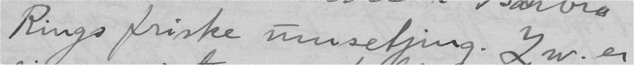Rings friske umsetjing. Zw. er
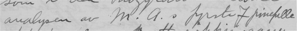analysen av M.A.s fyrste pinefulle
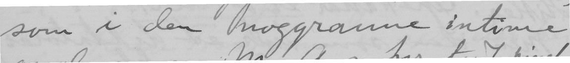som i den noggranne intime
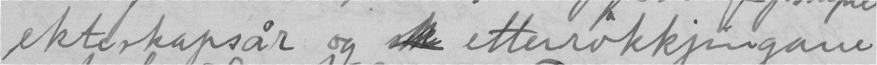ekteskapsår og  ettersökkjingane
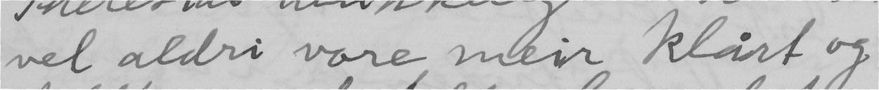vel aldri vore meir klårt og
